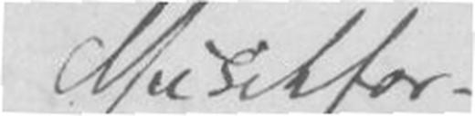Musikfor-
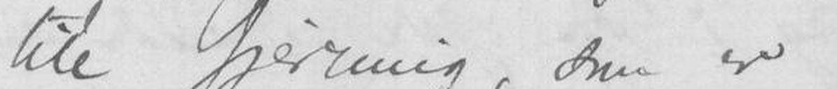tile Gjerning, som er
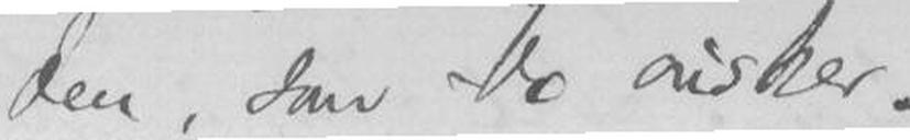den, som de ønsker.
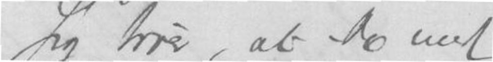Jeg tror, at De med
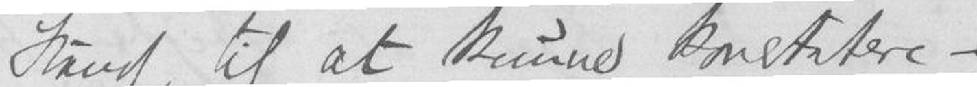stand til at kunde konstatere-
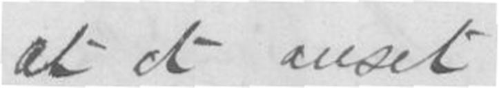at et anset
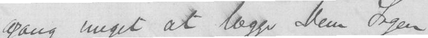gang meget at lægge Dem Sagen
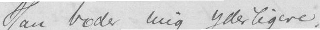Han boder mig yderligere
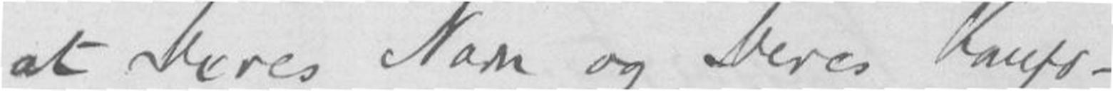at Deres og Deres -
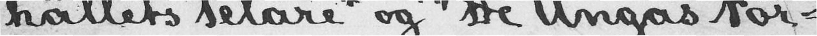hällets Pelare» og «De Ungas För-
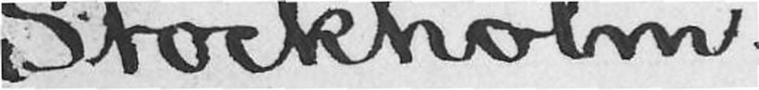Stockholm.
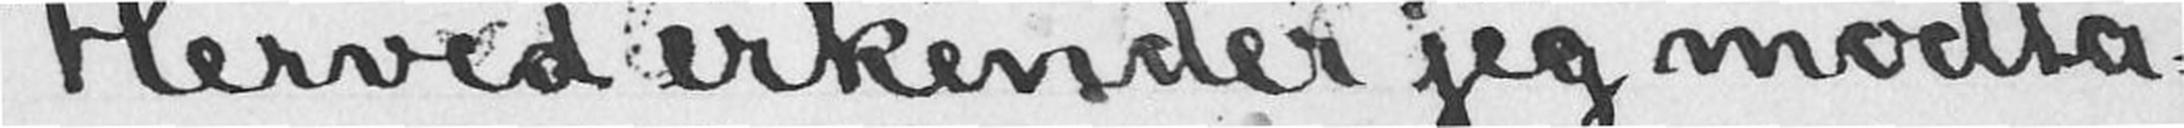Herved erkender jeg modta-
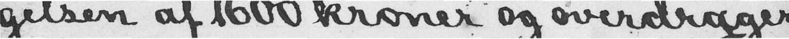gelsen af 1600 kroner og overdrgager
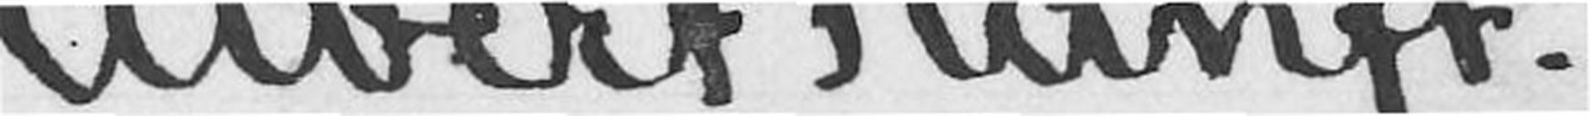Albert Ranft.
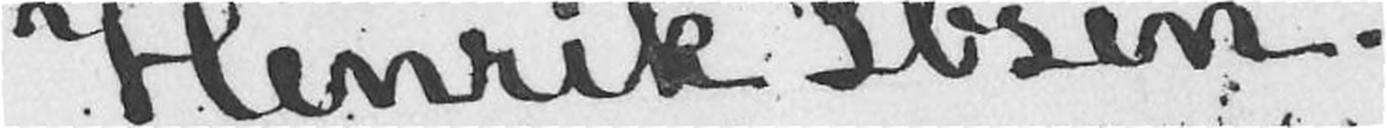Henrik Ibsen.
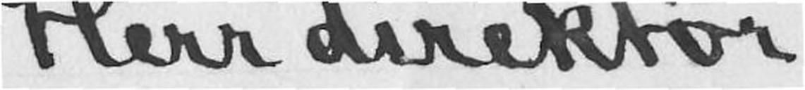Herr direktør
